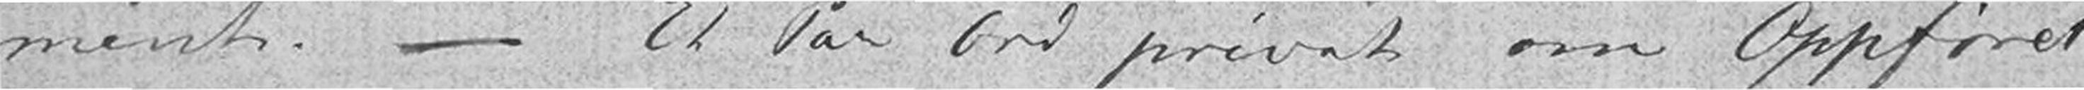ment.- Et Par Ord privat om Oppførel
